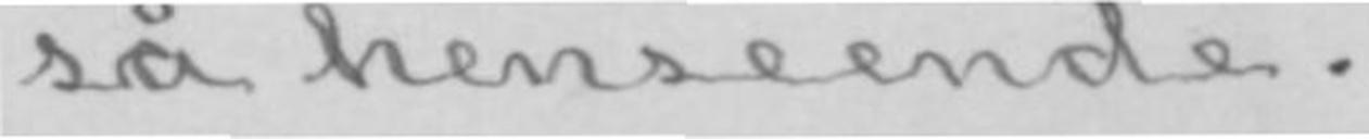så henseende.
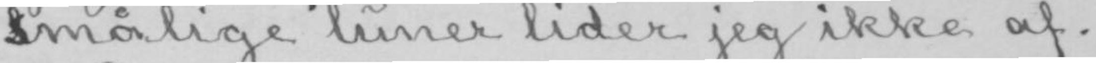smålige luner lider jeg ikke af.
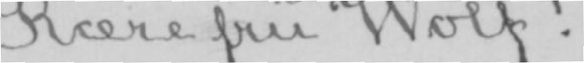Kære fru Wolf!
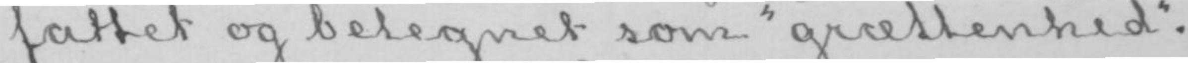fattet og betegnet som «grættenhed».
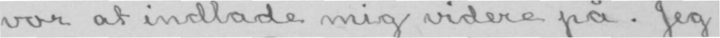vor at indlade mig videre på. Jeg
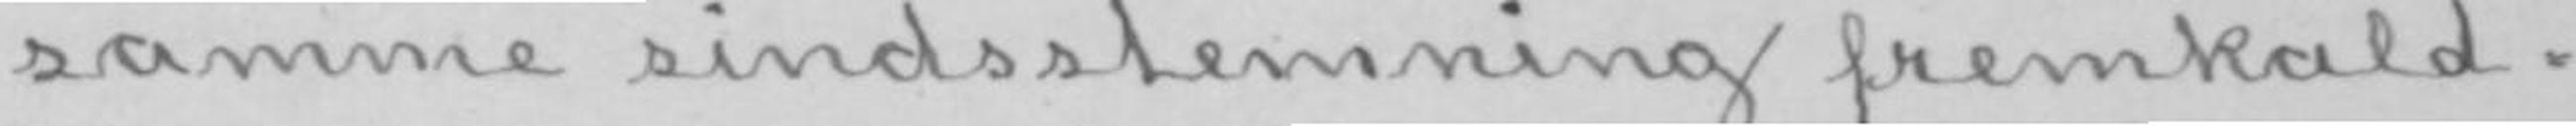samme sindsstemning fremkald-
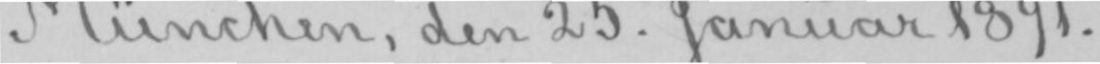München, den 25. Januar 1891.
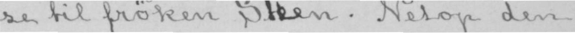se til frøken Steen. Netop den
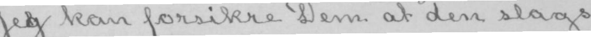Jeg kan forsikre Dem at den slags
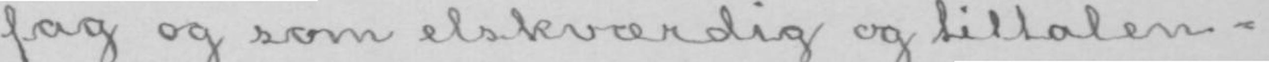fag og som elskværdig og tiltalen-
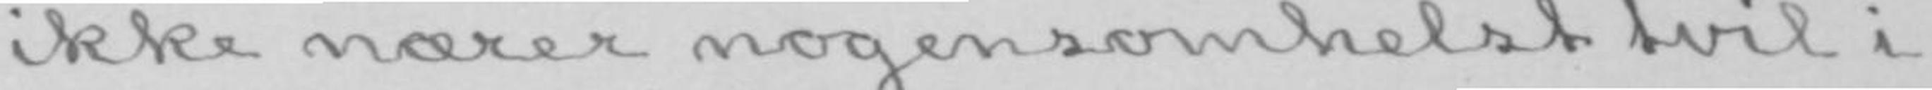ikke nærer nogensomhelst tvil i
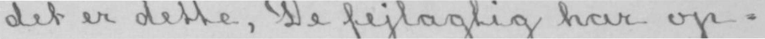det er dette, De fejlagtig har op-
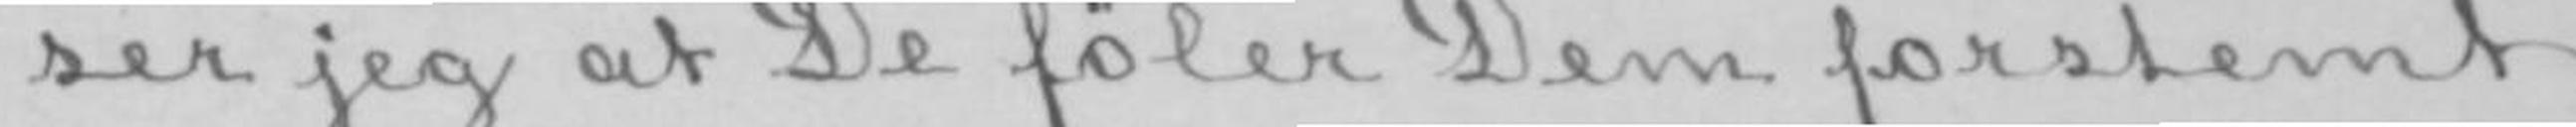ser jeg at De føler Dem forstemt
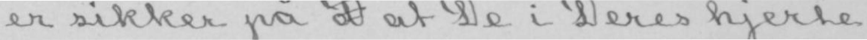er sikker på D at De i Deres hjerte
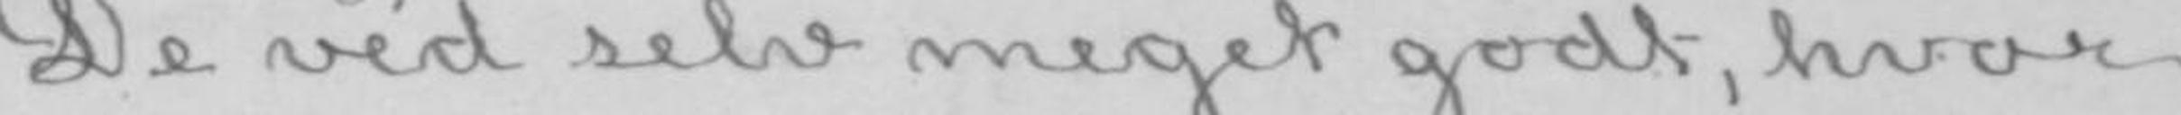De véd selv meget godt, hvor
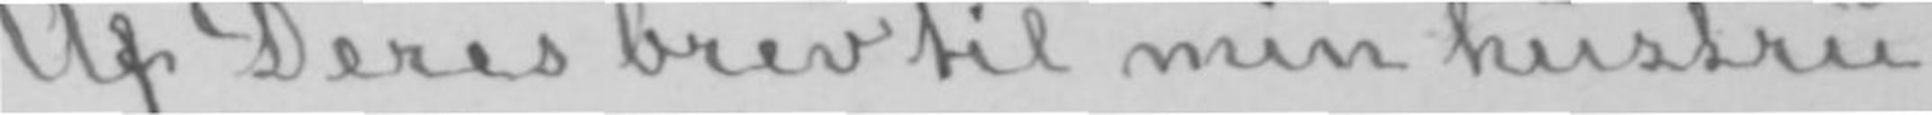Af Deres brev til min hustru
